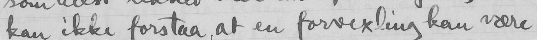kan ikke forstaa, at en forvexling kan være
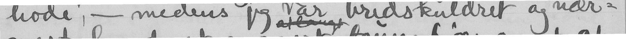hode, - medens jeg var bredskuldret og nær-
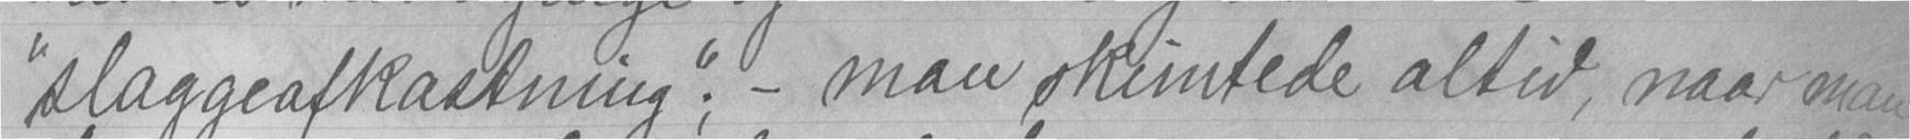"slaggeafkastning"; - man skimtede altid, naar man
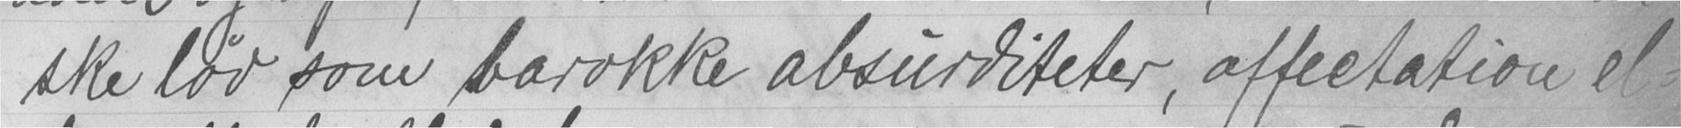ske lød som barokke absurditeter, affectation el-
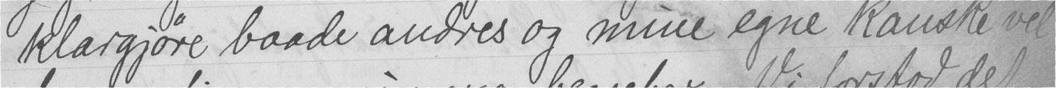klargjøre baade andres og mine egne kanske vel
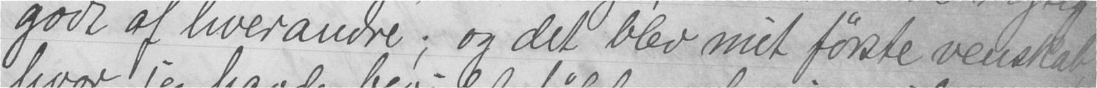godt af hverandre; og det blev mit første venskab
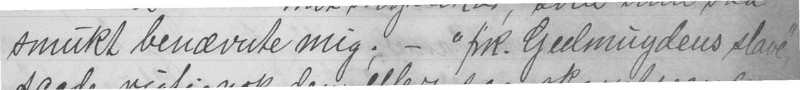smukt benævnte mig; - "frk. Geelmuydens slave,"
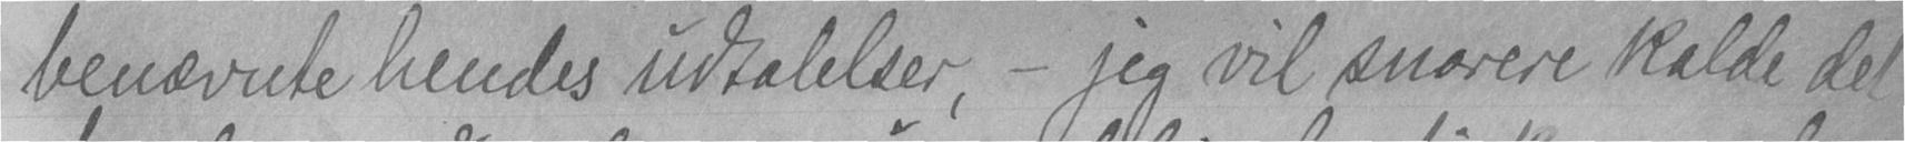benævnte hendes udtalelser, - jeg vil snarere kalde det
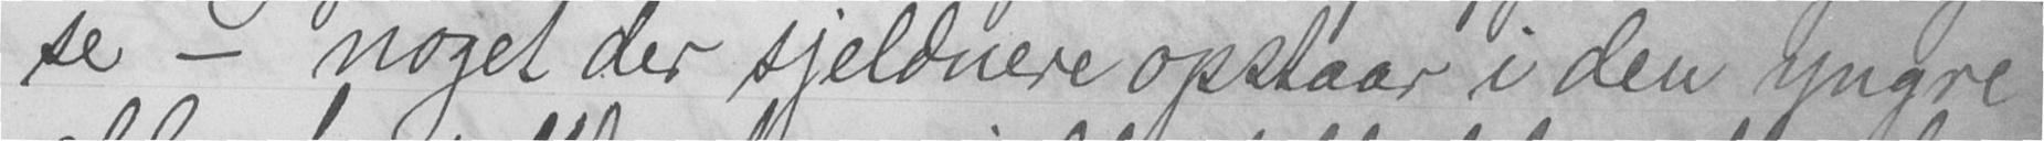se - noget der sjeldnere opstaar i den yngre
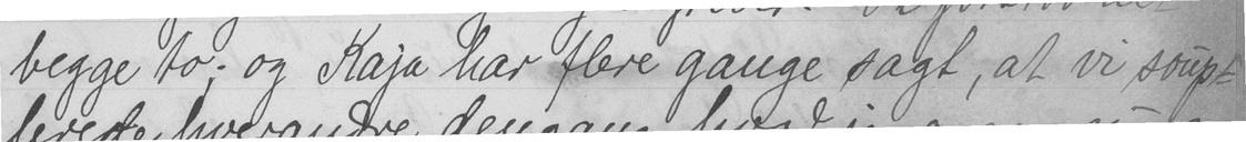begge to; og Kaja har flere gange sagt, at vi soup-
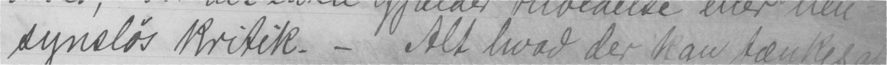synsløs kritik. - Alt hvad der kan tænkes af
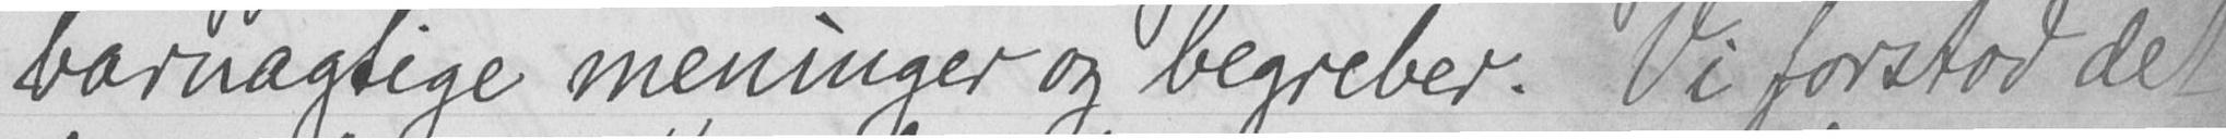barnagtige meninger og begreber. Vi forstod det
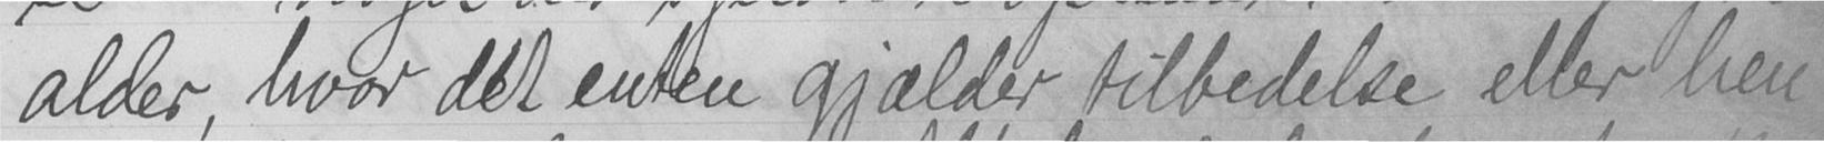alder, hvor det enten gjælder tilbedelse eller hen-
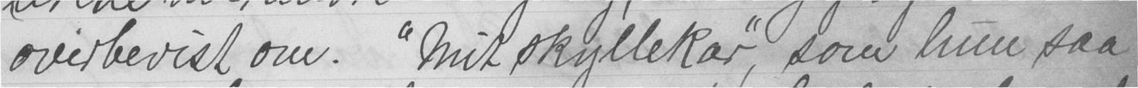overbevist om. "Mit skyllekar", som hun saa
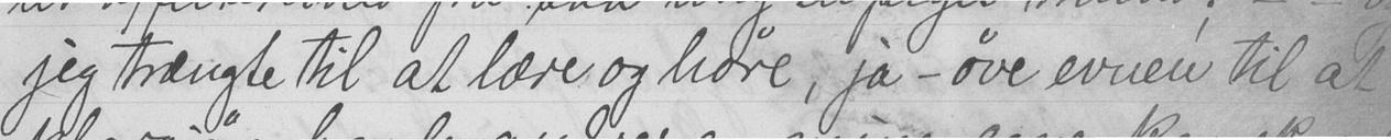jeg trængte til at lære og høre, ja - øve evnen til at
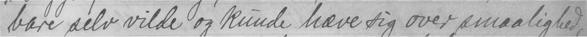bare selv vilde og kunde hæve sig over smaalighed,
